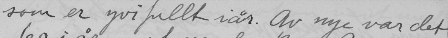som er yvifullt iår. Av nye var det
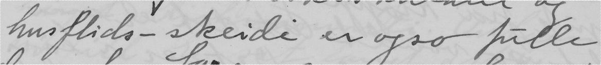husflids-skeide er ogso fulle
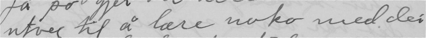utveg til å lære noko med dei
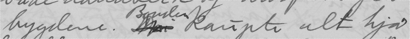bygdene. Bonden kaupte alt hjå
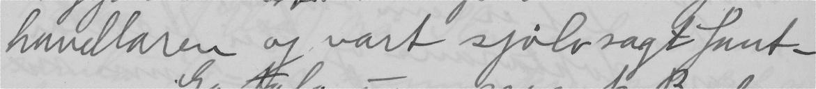handlaren og vart sjölvsagt fant –
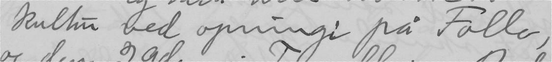kultu ved opningi på Follo,
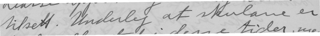tilsett. Underleg at skulane er
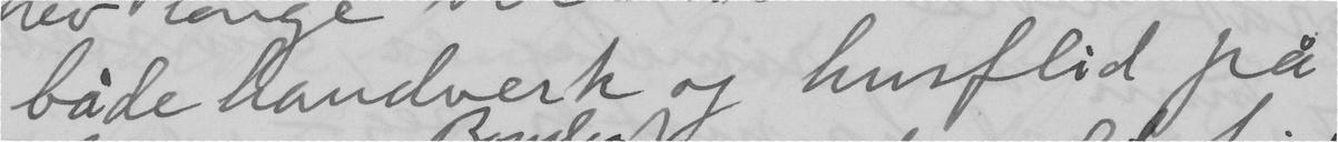både handverk og husflid på
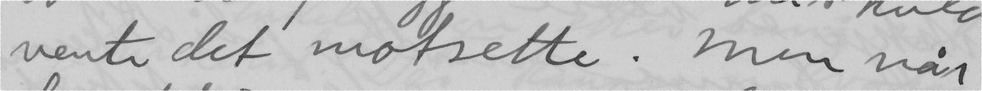vente det motsette. Men når
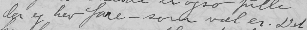der eg hev fare – som væl er. Det
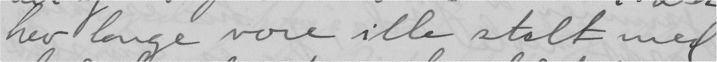hev lenge vore ille stelt med
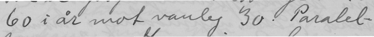60 i år mot vanleg 30. Paralel-
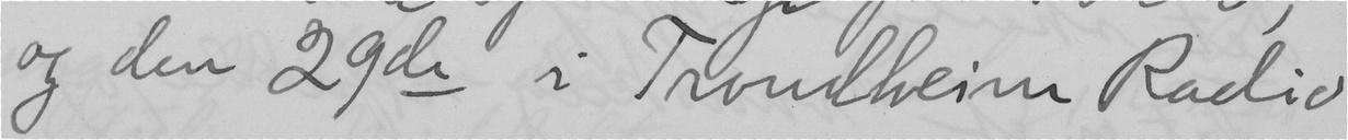og den 29de i Trondheim Radio
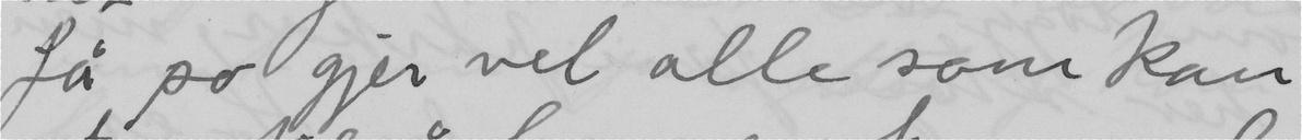få so gjer vel alle som kan
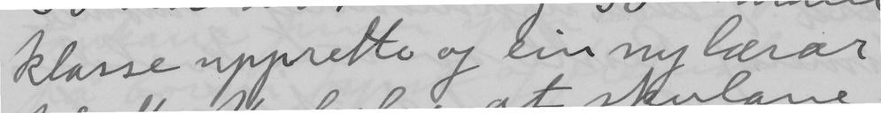klasse upprette og ein ny lærar
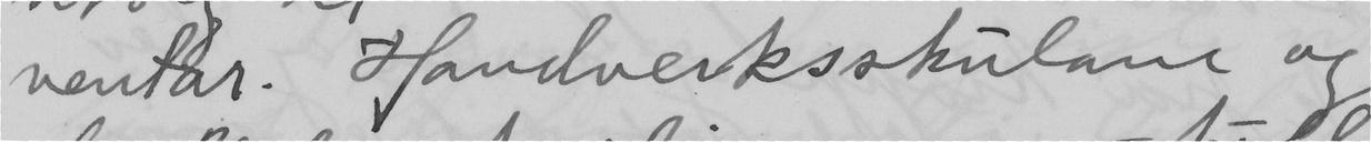ventar. Handverksskulane og
